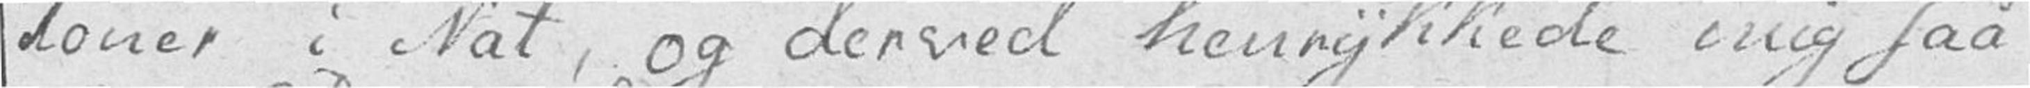Toner i Nat, og derved henrykkede mig saa
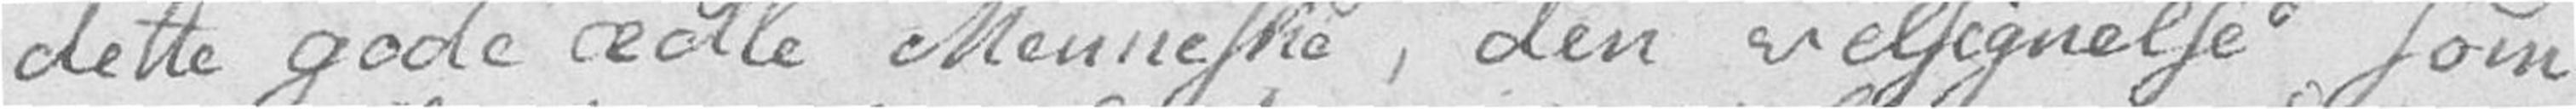dette gode ædle Menneske, den velsignelse som
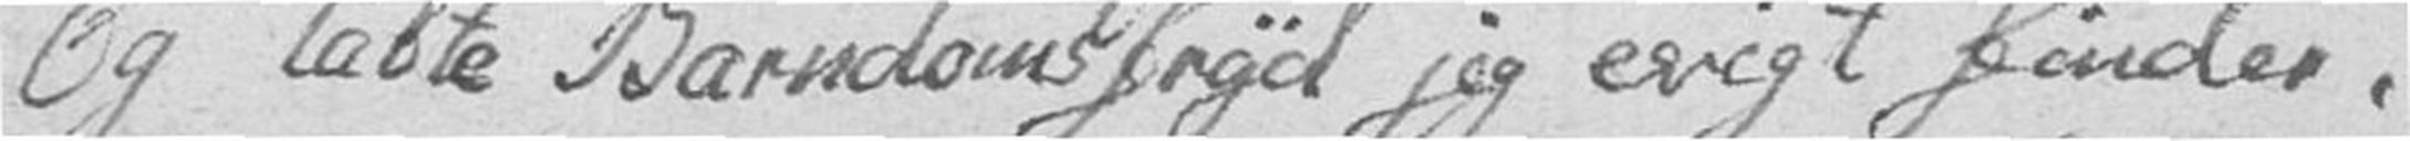Og tabte Barndomsfryd jeg evigt finder.
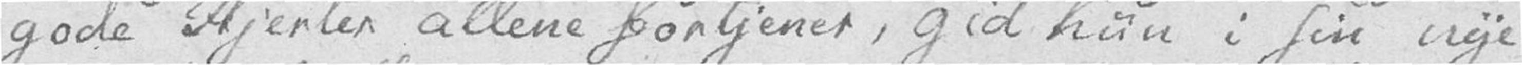gode Hjerter allene fortjener, gid hun i sin nye
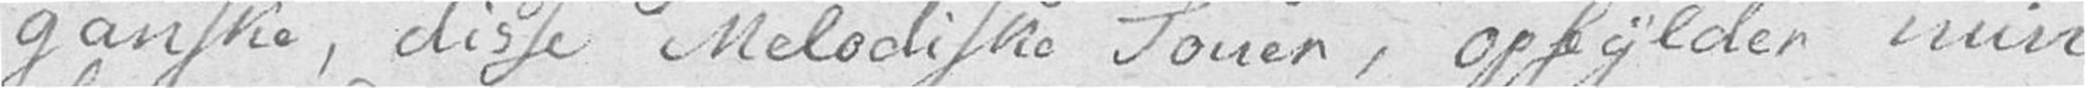ganske, disse Melodiske Toner, opfylder min
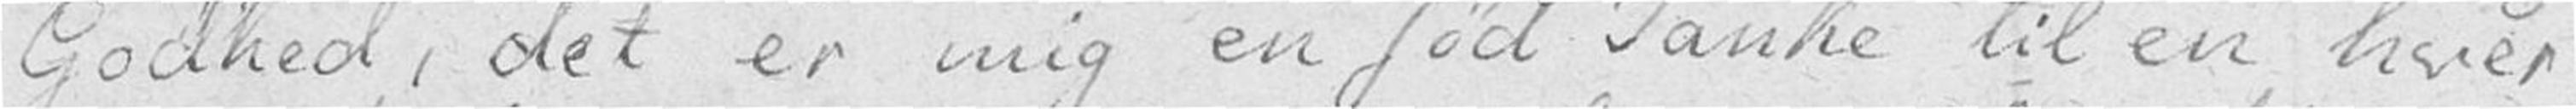Godhed, det er mig en sød Tanke til en hver
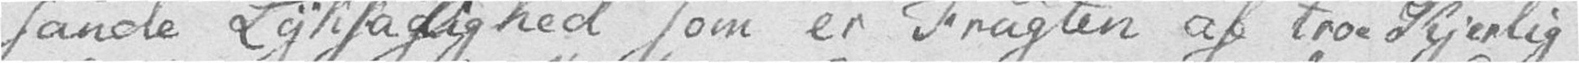sande Lyksalighed som er Frugten af troe Kjerlig
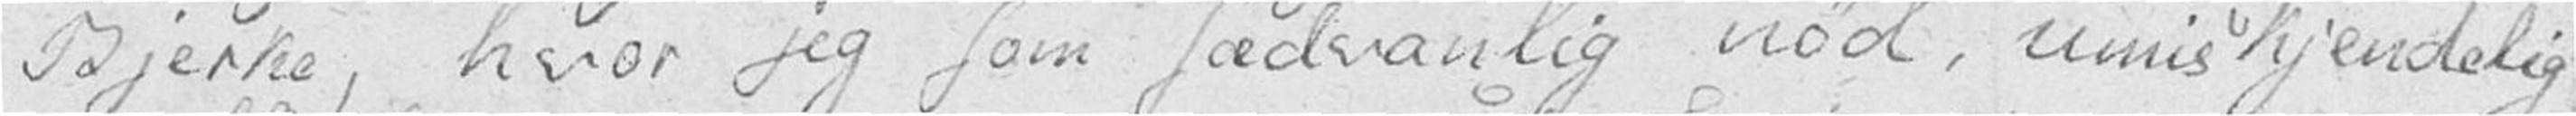Bjerke, hvor jeg som sædvanlig nød, umiskjendelig
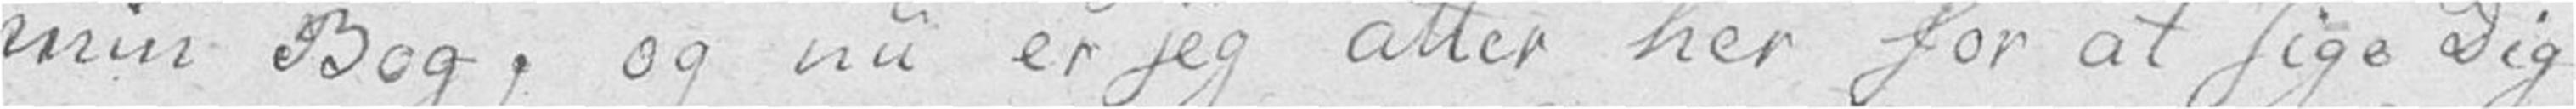min Bog, og nu er jeg atter her for at sige Dig
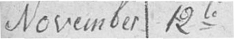November 12te
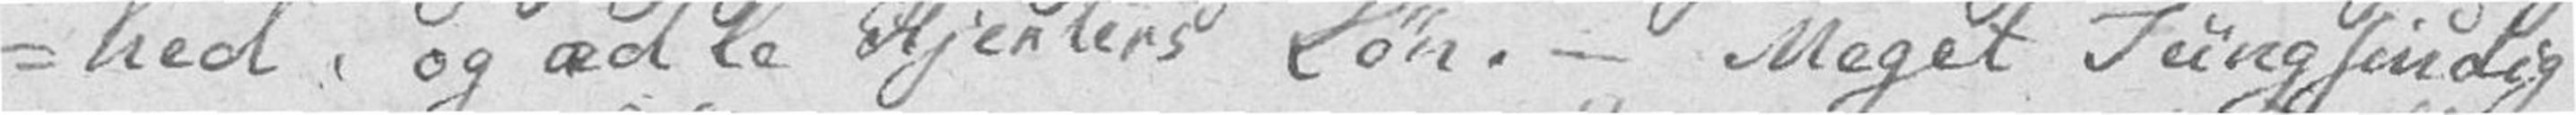-hed, og ædle Hjerters Løn. – Meget Tungsindig
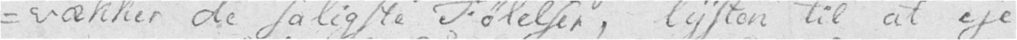-vækker de saligste Følelser, lysten til at eje
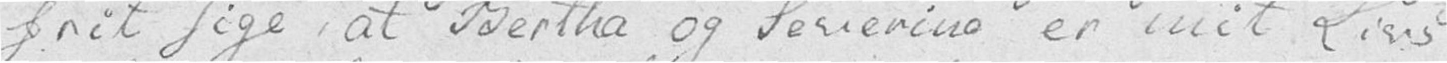frit sige, at Bertha og Severine er mit Livs
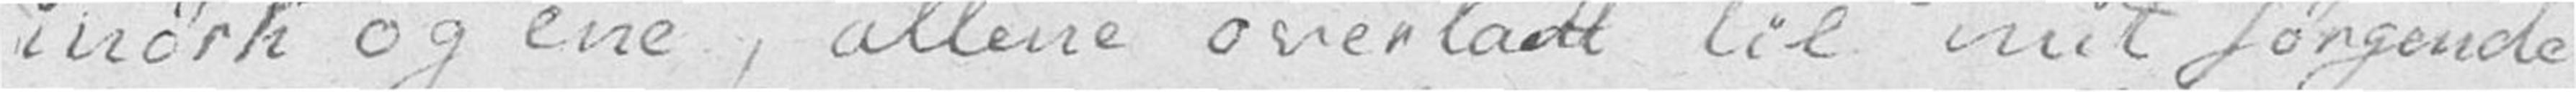mørk og ene, allene overladt til mit sørgende
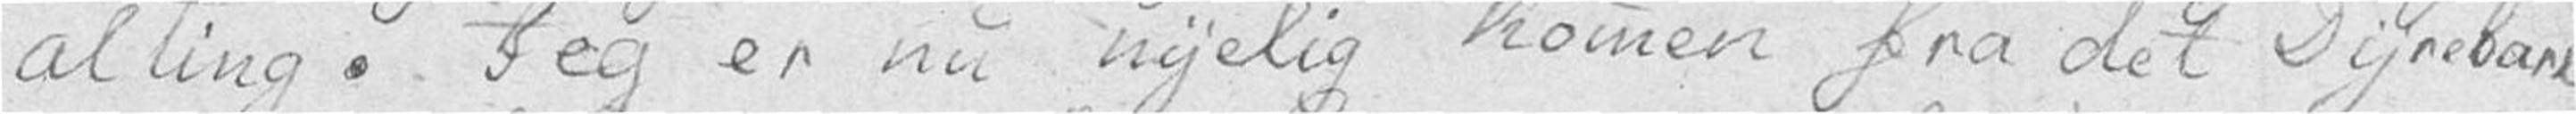alting. Jeg er nu nyelig kommen fra det Dyrebare
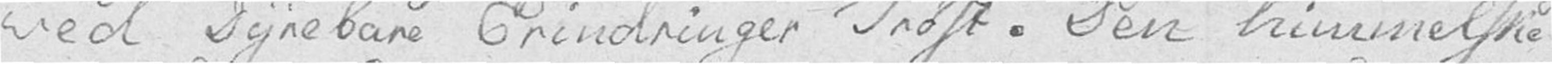ved Dyrebare Erindringer Trøst. Den himmelske
